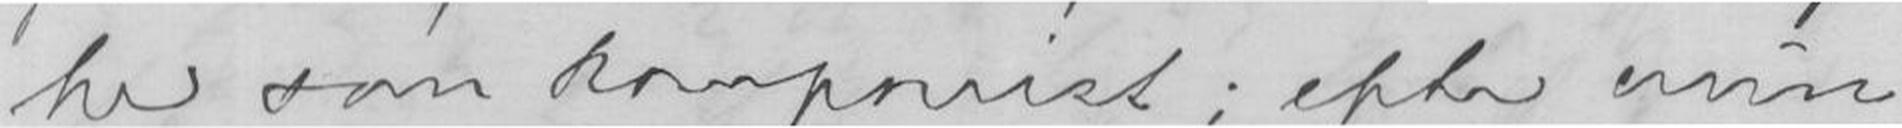her som komponist; efter min
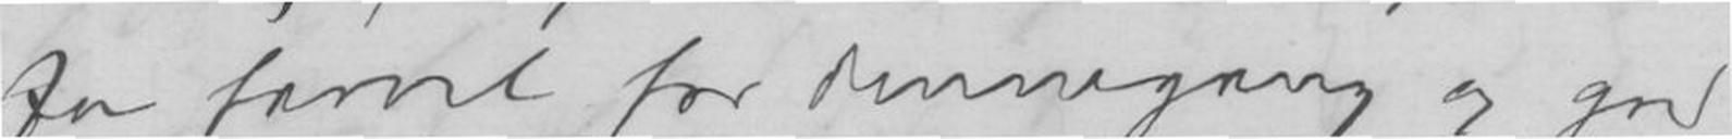Ja farvel for dennegang og god
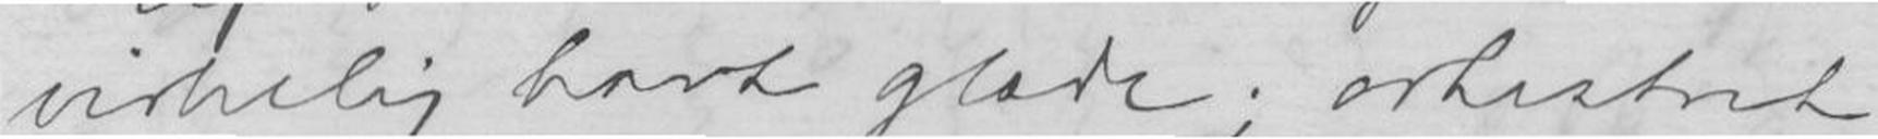virkelig havt glæde; orkestret
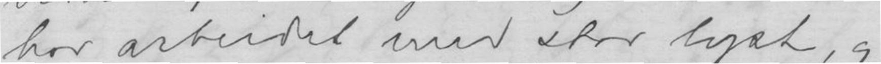har arbeidet med stor lyst, og
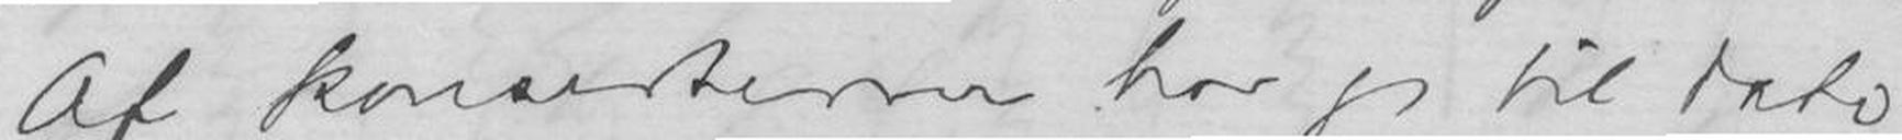Af konserterne har jeg til dato
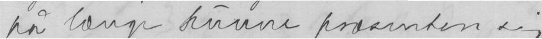på længe kunne præsentere sig
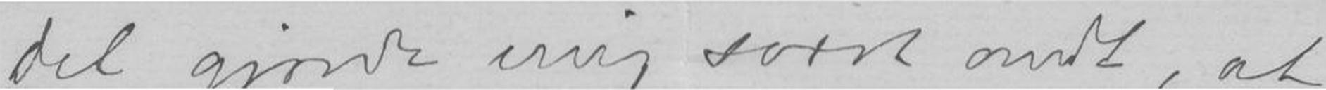Det gjorde mig svært ondt, at
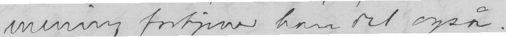mening fortjener han det også.
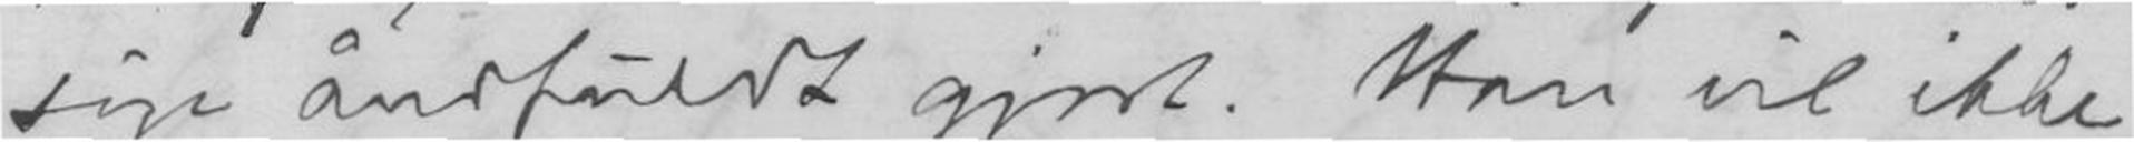sige åndfuldt gjort. Han vil ikke
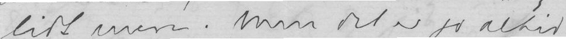lidt mere. Men det er jo altid
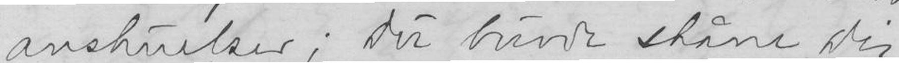anskuelser; du burde skåne dig
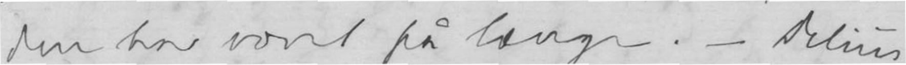den har været på længe.- Delius
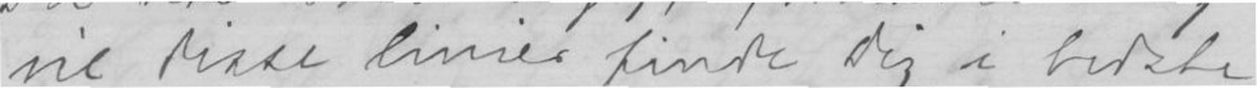vil disse linier finde dig i bedste
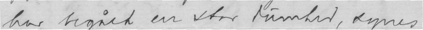har begået en stor dumhed, synes
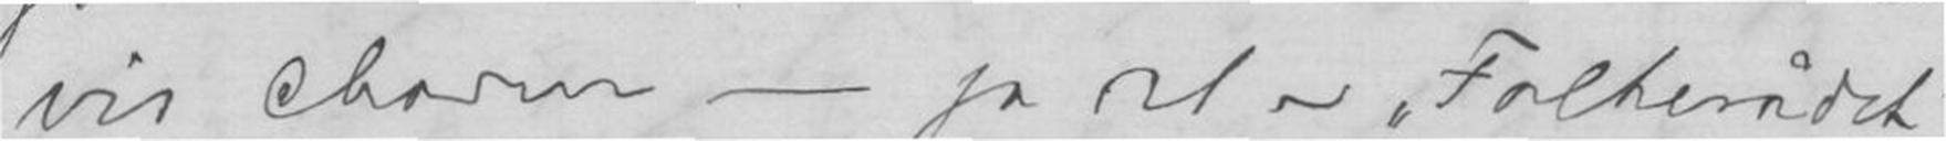vis charm - ja det er "Folkerådet
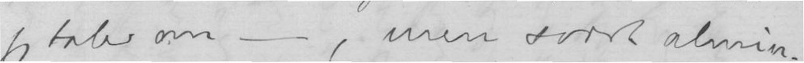jeg taler om -, men svært almin
